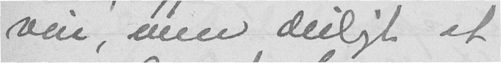veir, men deiligt at
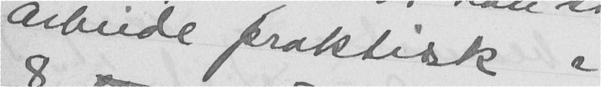arbeide praktisk i
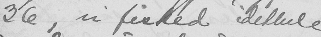36, vi fisked idethele
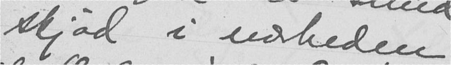skjød i nærheden
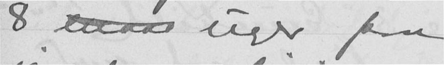8 maa uger paa
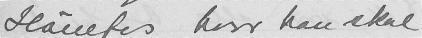Hønefos, hvor han skal
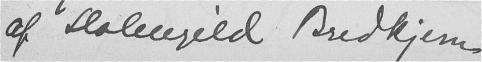af Holmegild Bredkjern
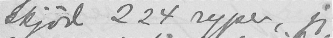skjød 224 ryper, jeg
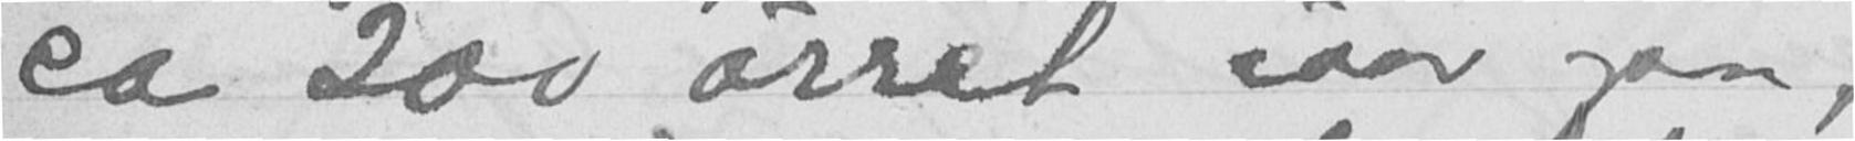ca 200 ørret iaar ogsaa,
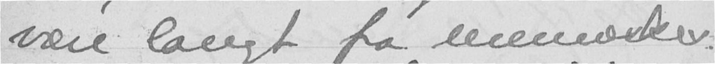være langt fra mennesker.
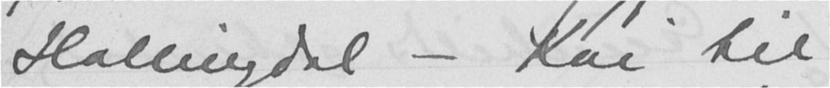Hallingdal - Kai til
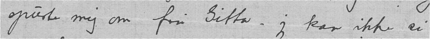spurte mig om fru Gitta – jeg kan ikke si
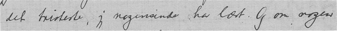det tristeste, jeg nogensinde har læst. Og om nogen
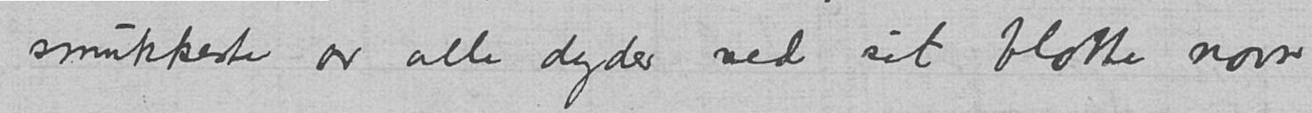smukkeste av alle dyder ved sit blotte navn
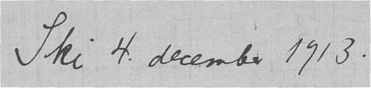Ski 4. december 1913.
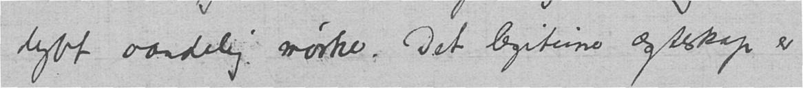dybt aandelig mørke. Det legitime ægteskap er
